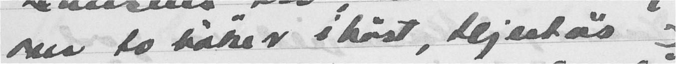over to bøker ihøst, Hjertøs og
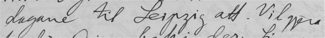dagane til Leipzig att. Vil gjera
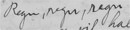Regn, regn, regn –
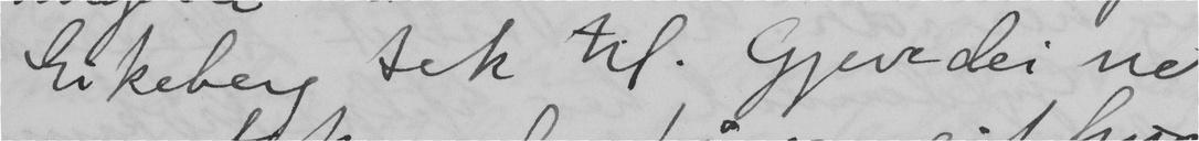Eikeberg tek til. Gjeve dei no
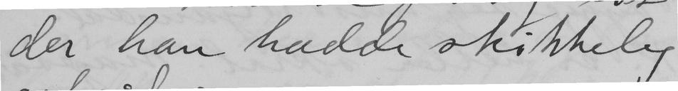der han hadde skikkeleg
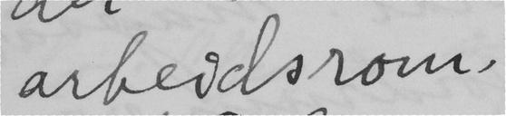arbeidsrom.
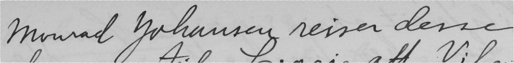Monrad Johansen reiser desse
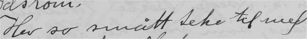Hev so smått teke til med
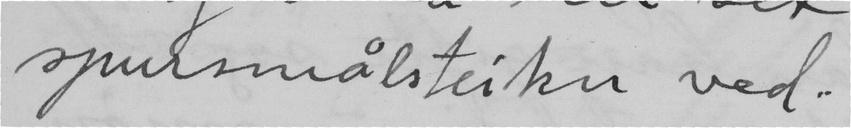spursmålsteikn ved.
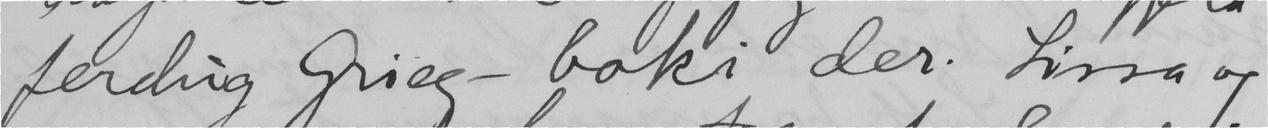ferdig Grieg-boki der. Lissa og
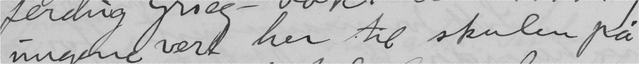ungene vert her til skulen på
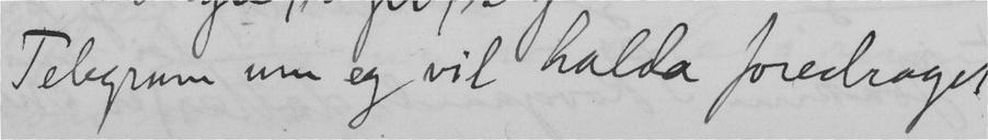Telegram um eg vil halda foredraget
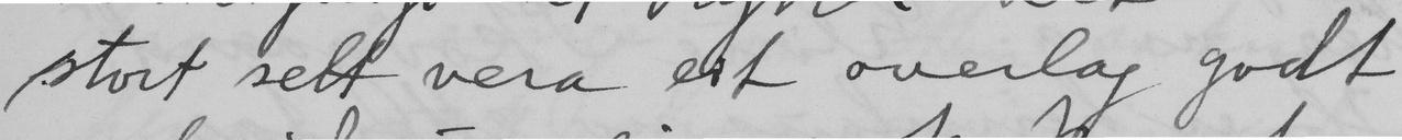stort sett vera eit overlag godt
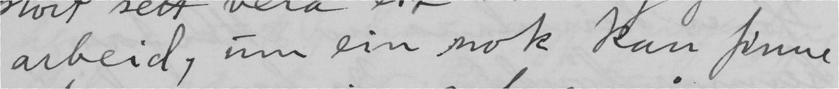arbeid, um ein nok kan finne
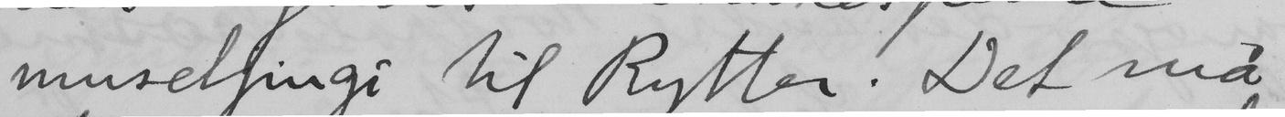muselfingi til Rytter. Det må
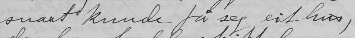snart kunde få seg eit hus,
